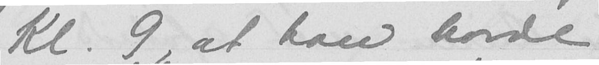kl. 9, at han havde
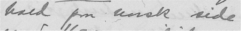hold paa norsk side
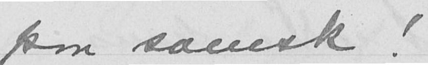paa svensk!
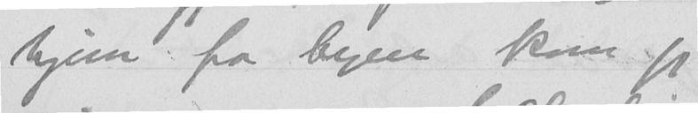hjem fra byen kom jeg
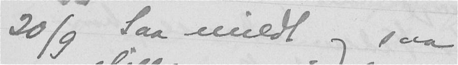20/9 Saa mildt og saa
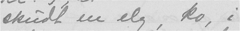skudt en elg, ko, i
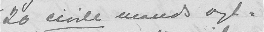20 civile mands vagt-
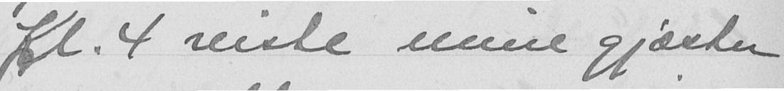Kl. 4 reiste mine gjæster
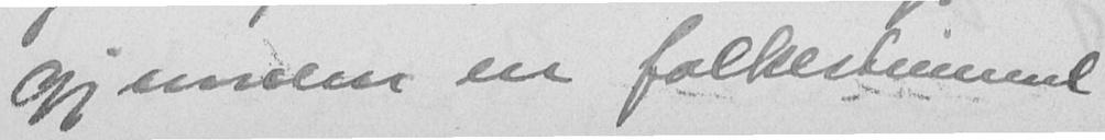gjennem en folkestimmel
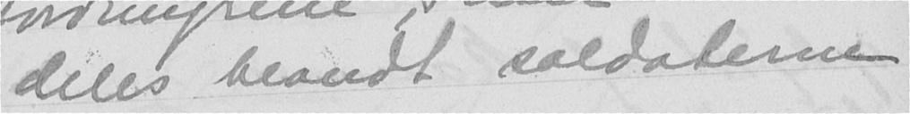deles blandt soldaterne
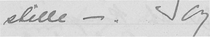stille -. Og
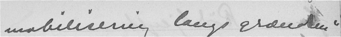mobilisering langs grændsen"
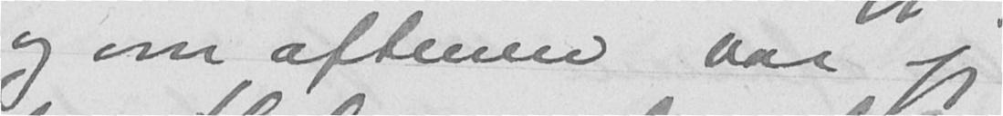og om aftenen var jeg
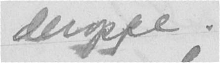deroppe.
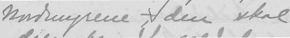Nordmyrene, - den skal
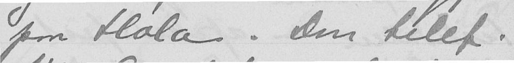paa Hola. Don telef.
